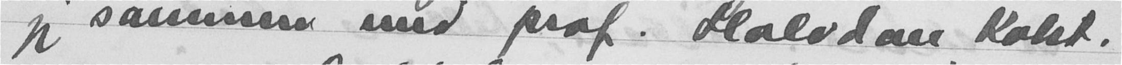jeg sammen med prof. Halvdan Koht.
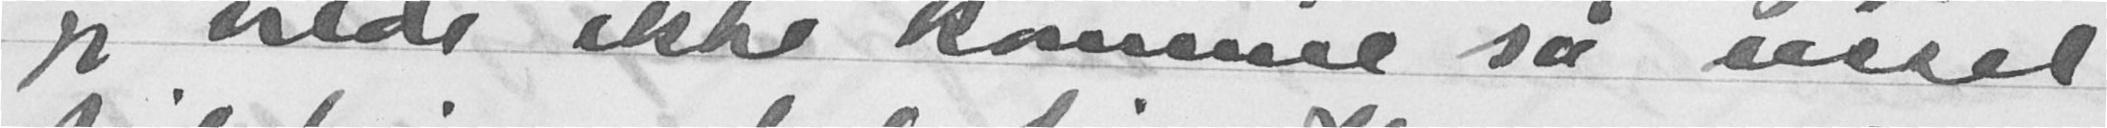jeg vilde ikke komme så ussel
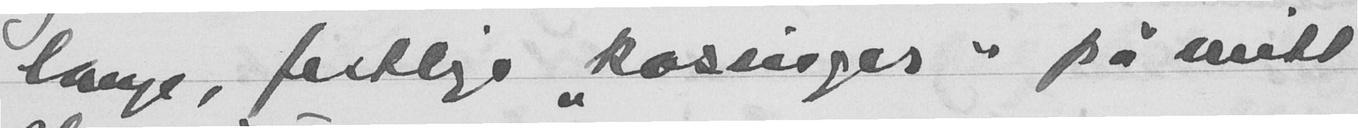lange, festlige "kosinger" på mitt
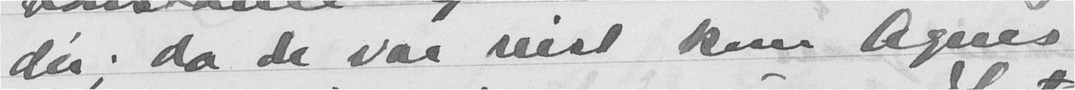der; da de var reist kom Agnes
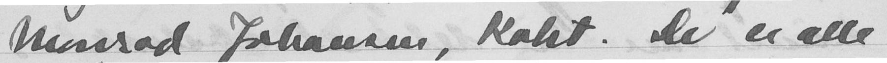Monrad Johansen, Koht. De er alle
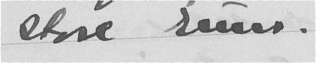store rum.
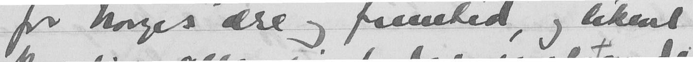for Norges ære og fremtid, og likevel
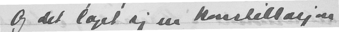Og det laget sig en konstellasjon
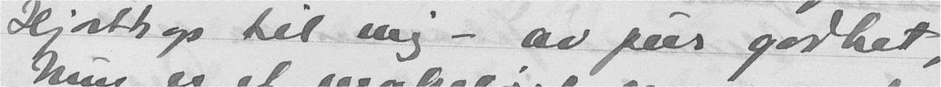Hjort op til mig - av pur godhet.
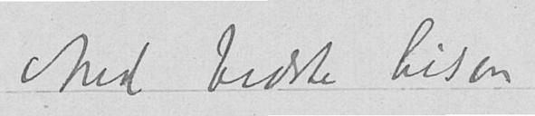Med bedste hilsen
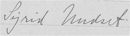Sigrid Undset.
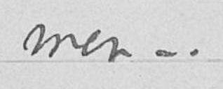men –.
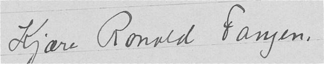Kjære Ronald Fangen,
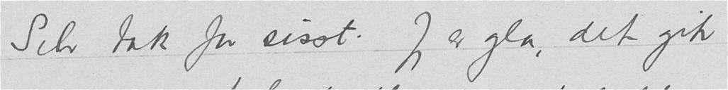Selv tak for sisst. Jeg er gla, det gik
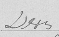Deres
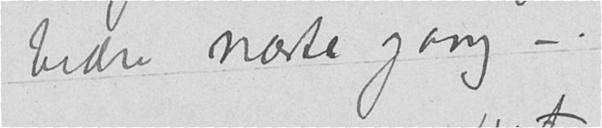bedre næste gang –.
